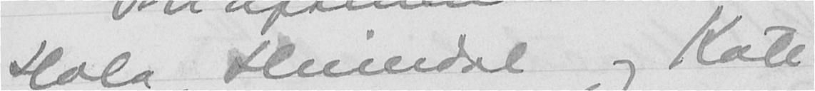Hola, Heimdal og Kate
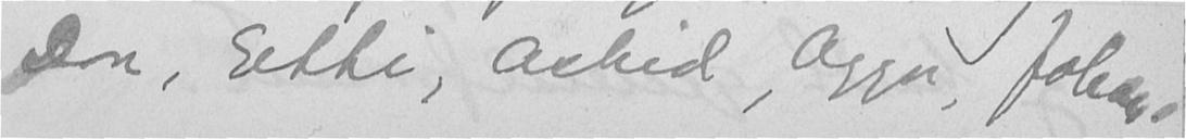Don, Etti, Astrid, Agga, Johan.
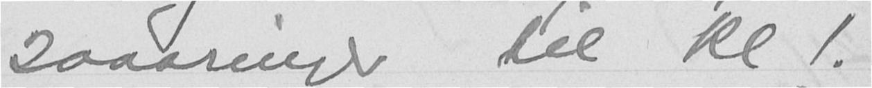20aaringer til kl 1.
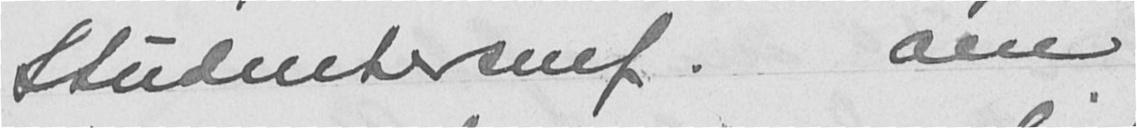Studentersmf. om
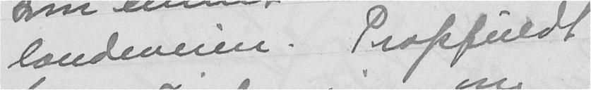landeveien. Propfuldt
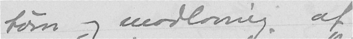børn og madlavning af
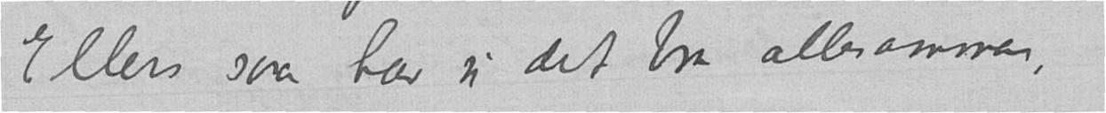Ellers saa har vi det bra allesammen,
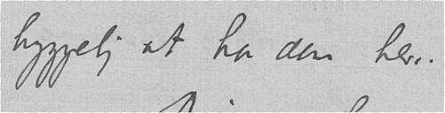hyggelig at ha dem her.
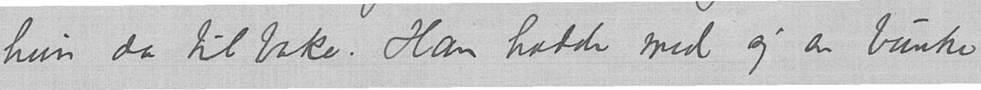hun da tilbake. Han hadde med sig en bunke
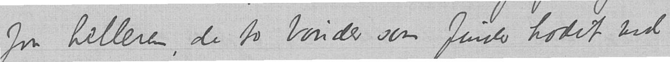fra helleren, de to bønder som finder hodet ved
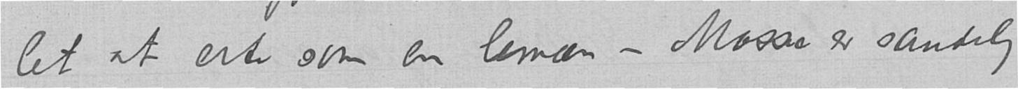let at erte som en lemæn – Mosse er sandelig
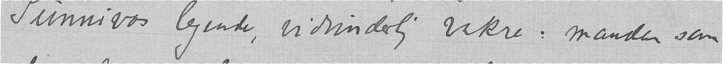Sunnivas legende, vidunderlig vakre: manden som
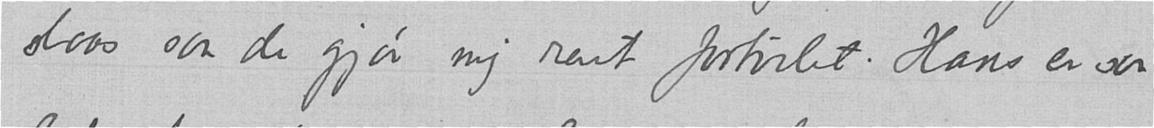sloss saa de gjør mig rent fortvilet. Hans er saa
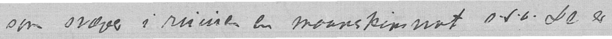som svæver i ruinen en maaneskinsnat o.s.v. De er
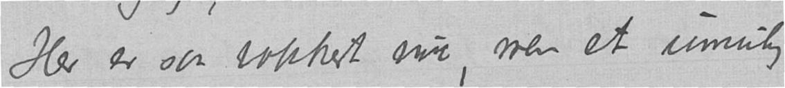Her er saa vakkert nu, men et umulig
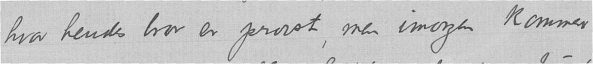hvor hendes bror er prost, men imorgen kommer
